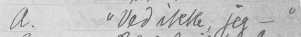A. "Ved ikke, jeg -"
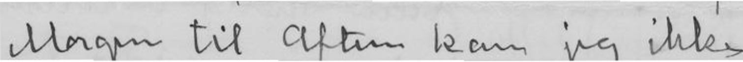Morgen til Aftens kan jeg ikke
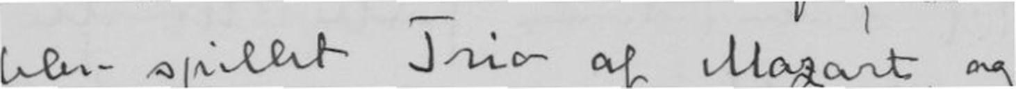blev spillet Trio af Mozart, og
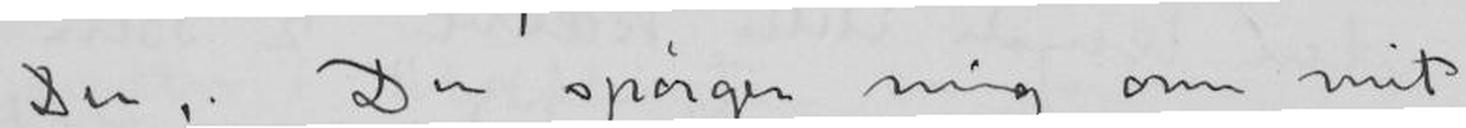Du, Du spørger mig om mit
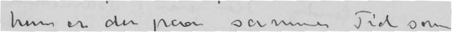hun er der paa samme Tid som
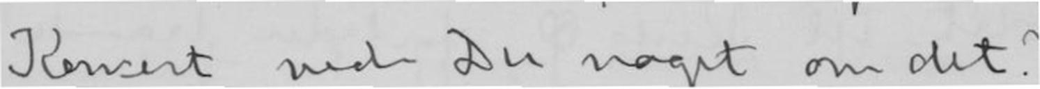Koncert, ved Du noget om det?
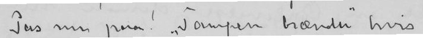Pas nu paa! "Tampen brænder" hvis
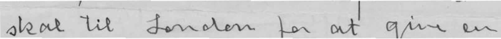skal til London for at give en
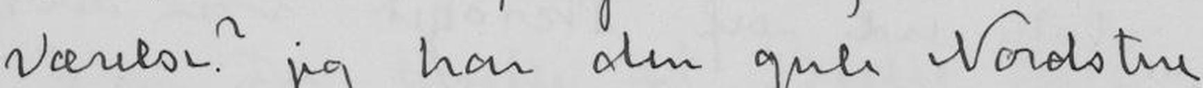Værelse? jeg har den gule Nordstue
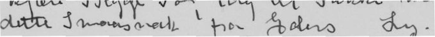dette Smaasnak fra Eder Ly.
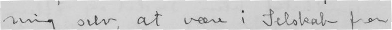mig selv, at være i Selskab fra
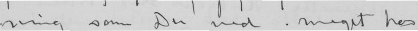mig som De ved meget hos
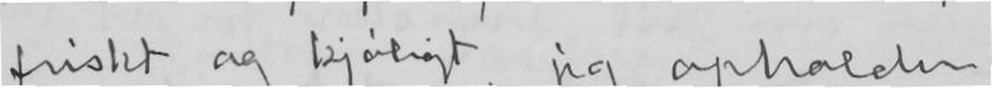friskt og kjøligt, jeg opholder
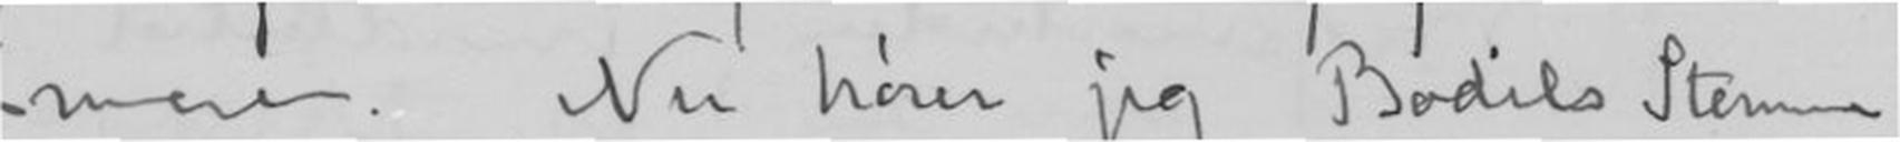mere. Nu hører jeg Bodils Stemme
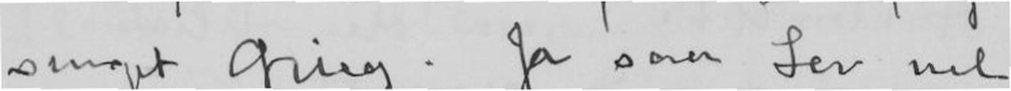sunget Grieg. Ja saa Lev vel
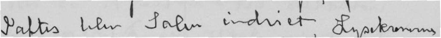Iaftens blev Salen indviet, Lysekronene
